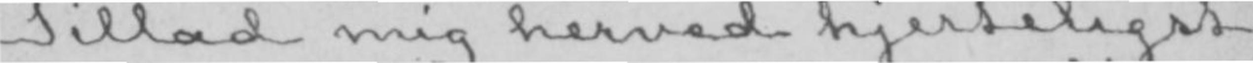Tillad mig herved hjerteligst
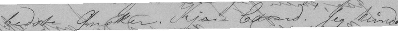bedste Ønsker. Kjære Edvard! Jeg kunde
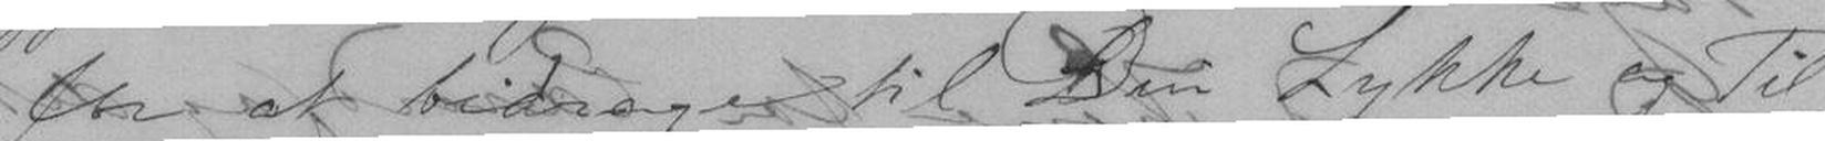for at bidraget til Din Lykke og Til
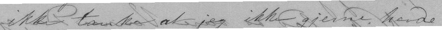ikke tænke at jeg ikke gjerne havde
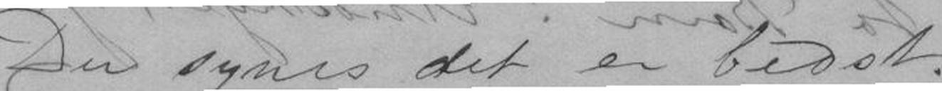Du synes det er bedst.
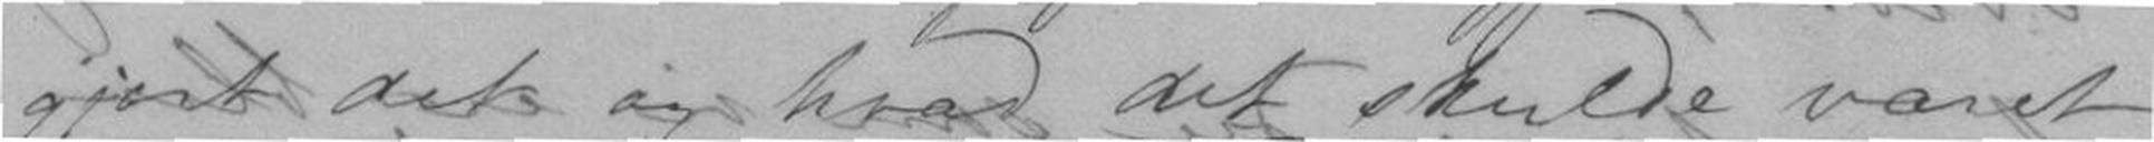gjort det og hvad det skulde været
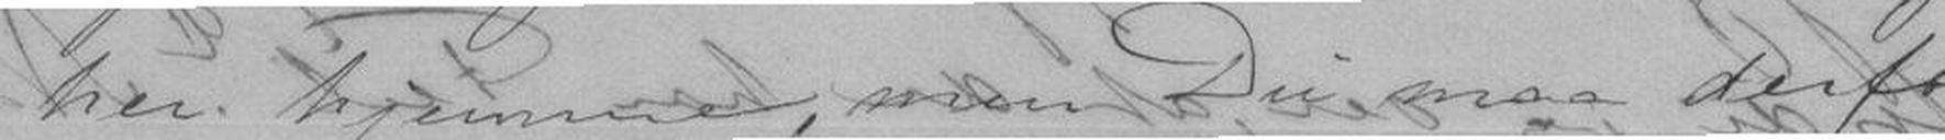her hjemme, men Du maa derfor
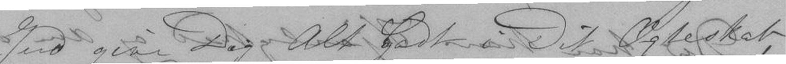Gud give Dig Alt Godt i Dit Ægteskab,
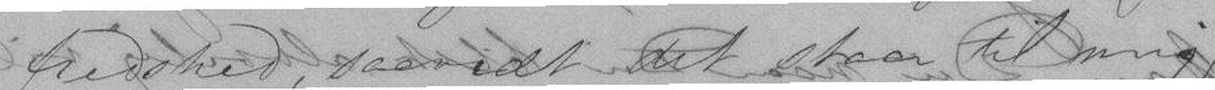frihed, saavidt det staar til mig;
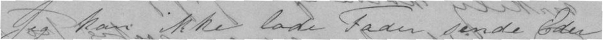Jeg kan ikke lade Fader sende Eder
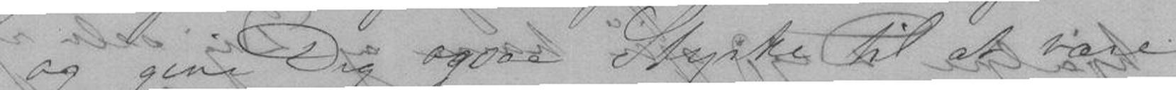og give Dig ogsaa Styrke til at være
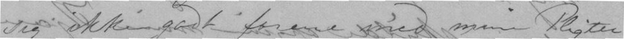sig ikke godt forene med mine Pligter
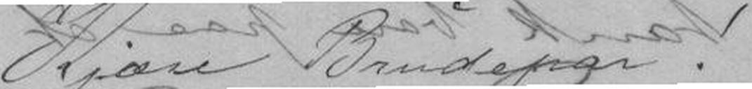Kjære Brudepar!
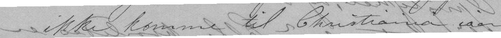ikke komme til Christiania iaar,
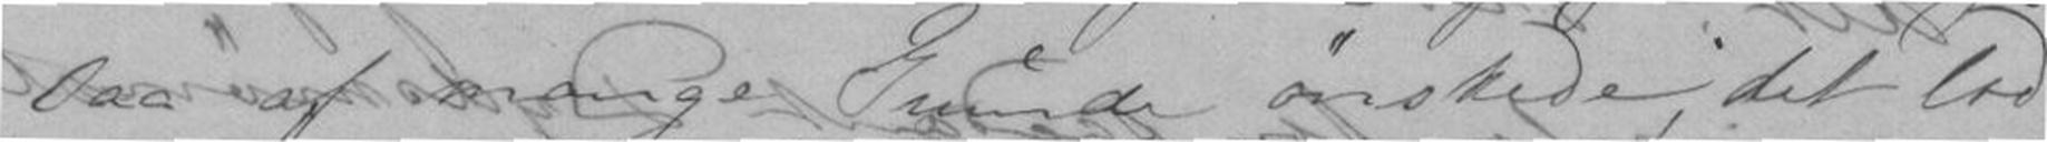saa af mange Grunde ønskede; det lod
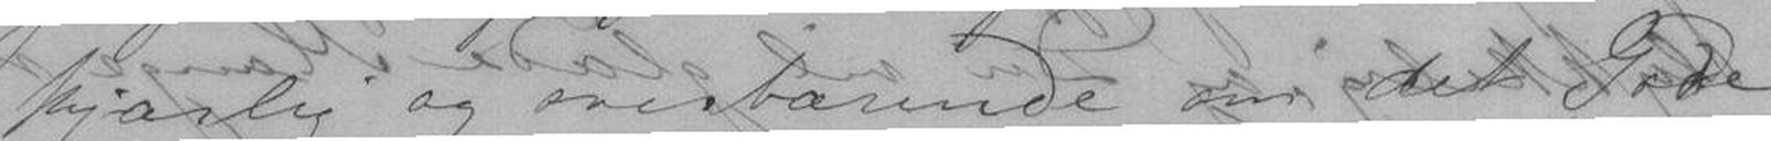hjærtelig og overbærende om det Gode
